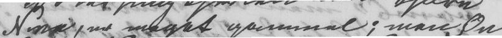Nina, er meget gammel; men On-
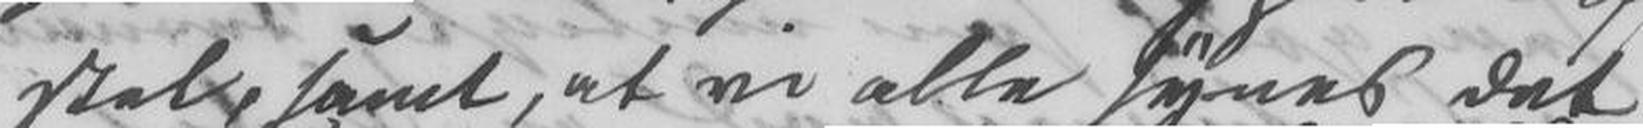stel, samt, at vi alle synes det
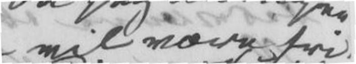vil være fri
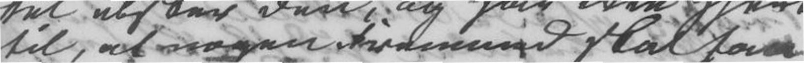til, at nogen Fremmed skal faa
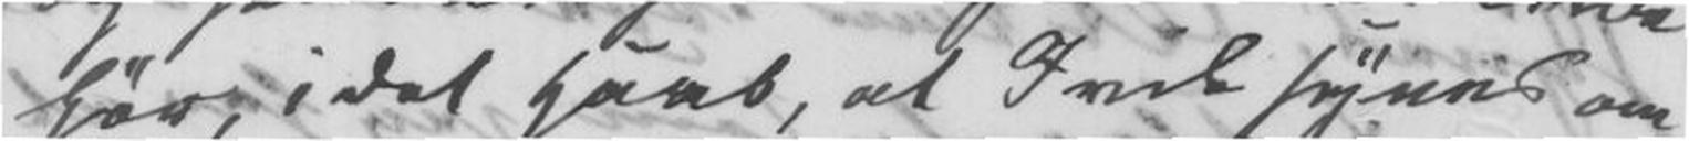hør; i det haab, at I vil synes om
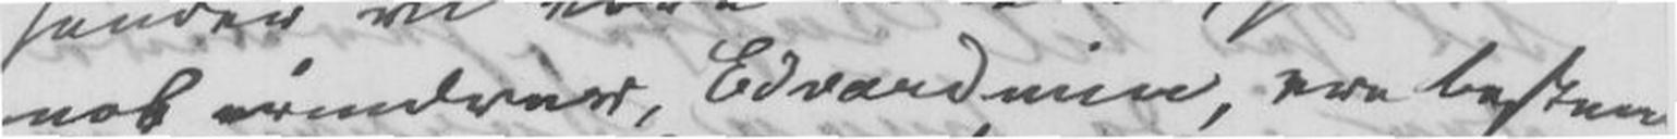nok erindrer, Edvard min, er besten
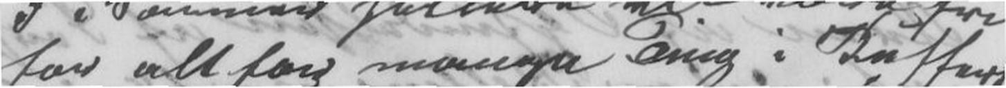for alt for mange Ting i Kuffert
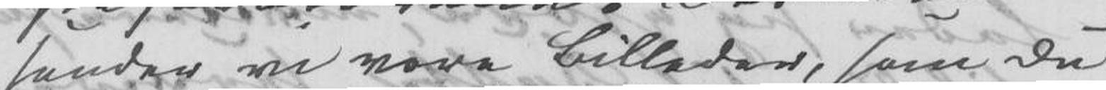saadan vi vore billeder, som Du
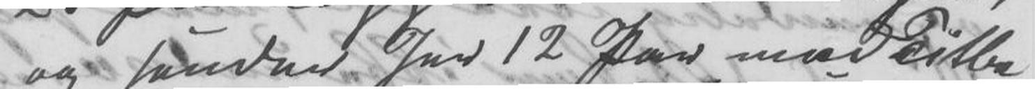og saadan Jer 12 Par med Tilbe
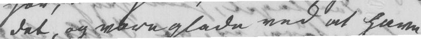det, og være glade ved at gang
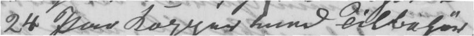14 Par kopper med Tilbehør,
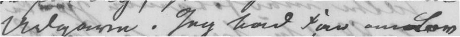Udgave. Jeg bad Far om Lov
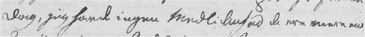Dag, jeg havde ingen Medlidenhed de ere mere end
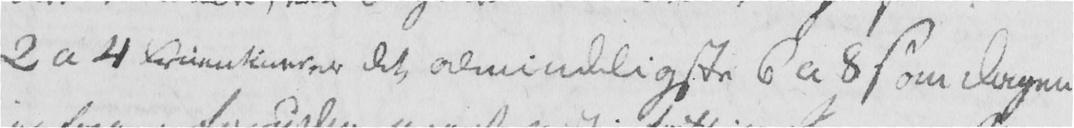2 a 4 Fruentimmer det almindeligste 6 a 8 s om dagen
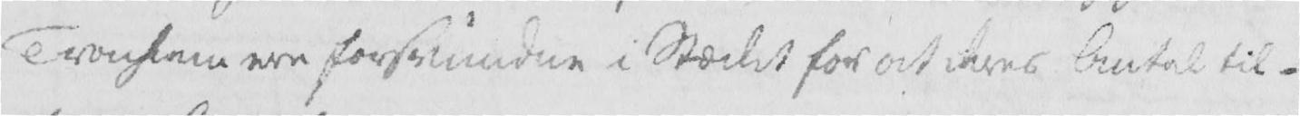Tronhiem ere forsvundne i Stædet for at deres Antal til-
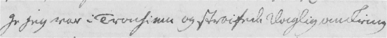ge jeg var i Tronhiem og streifede daglig omkring
